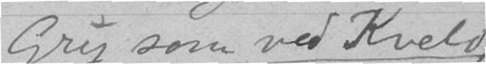Gry som ved Kveld,
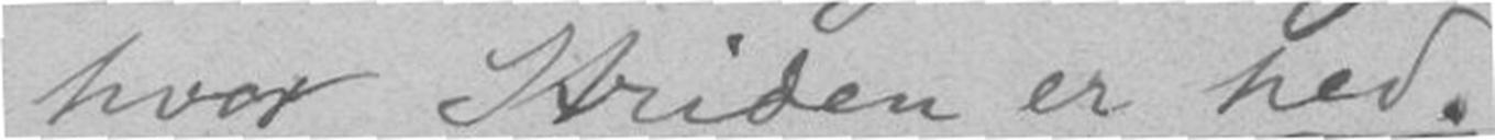hvor Striden er hed.
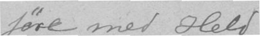føre med Held
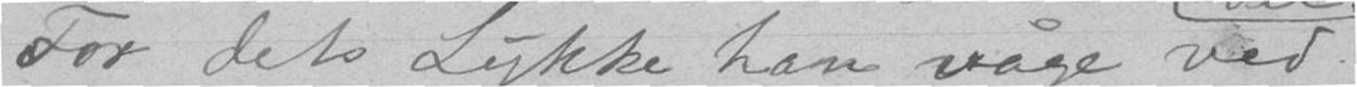For dets Lykke han våge ved
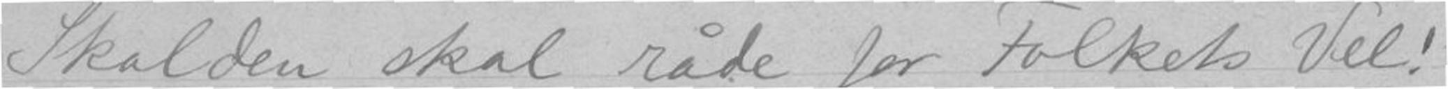Skalden skal råde for Folkets Vel!
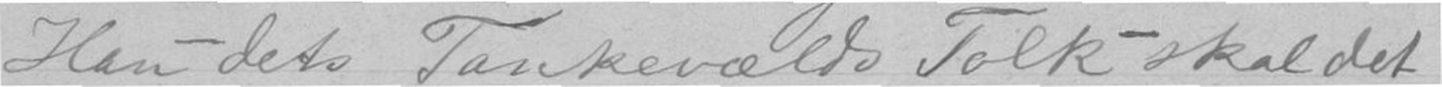Han - dets Tankevælds Folk - skal det
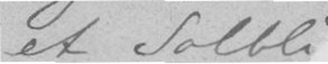et Solbli
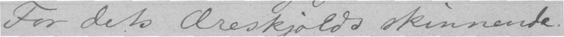For dets Æreskjolds skinnende
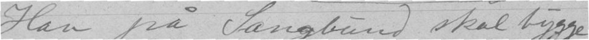Han på Sangbund skal bygge
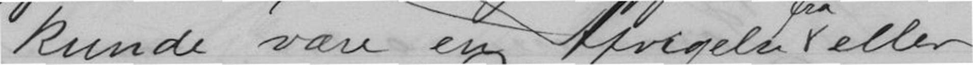kunde være en Afvigelse fra eller
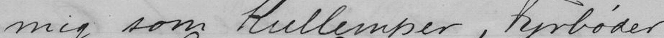mig som Kullemper, Fyrbøter
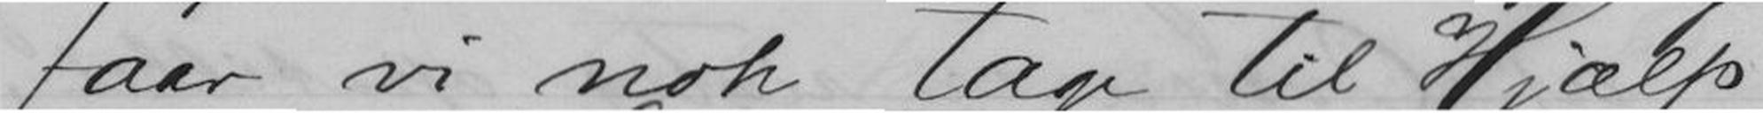faar vi nok tage til Hjælp
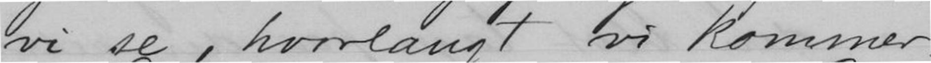vi se, hvorlangt vi kommer.
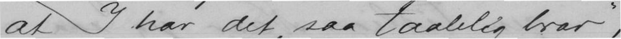at I har det "saa taalelig brav",
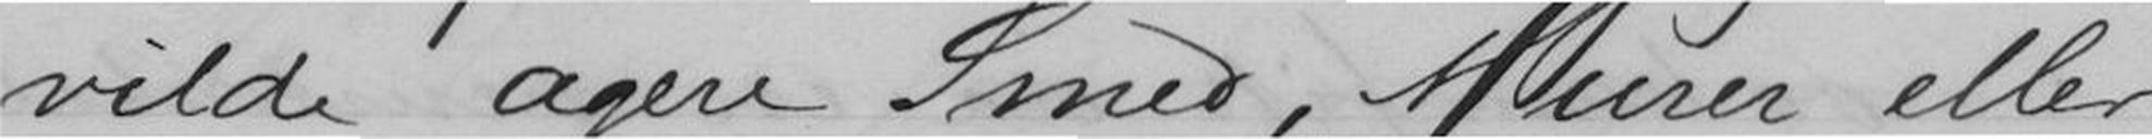vilde agere Smed, Murer eller
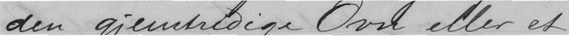den gjenstridige Ovn eller et
